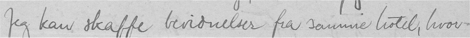Jeg kan skaffe bevidnelser fra samme hotel, hvor-
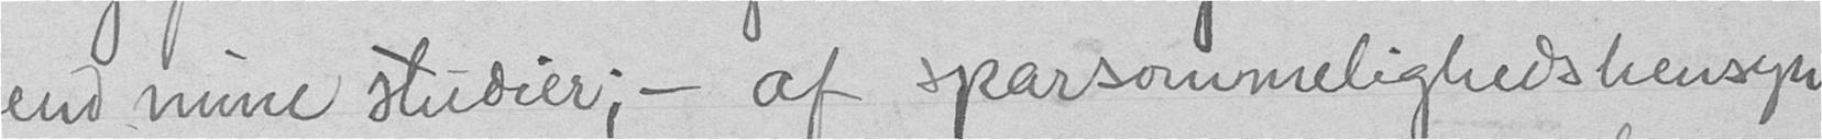end mine studier, - af sparsommelighedshensyn
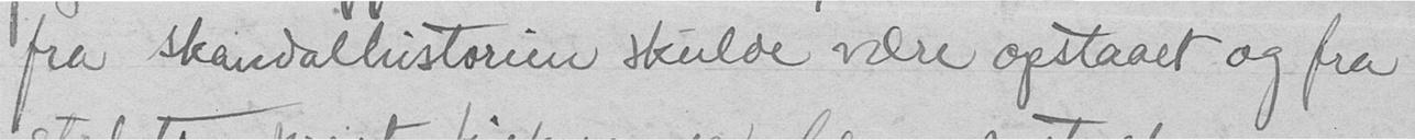fra skandalhistorien skulde være opstaaet og fra
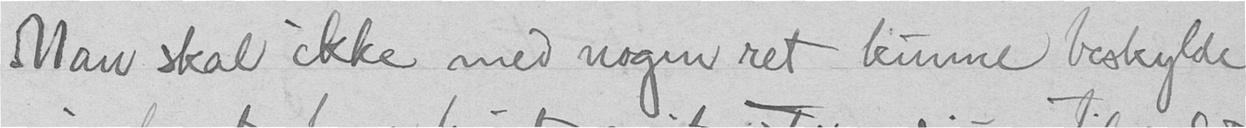Man skal ikke med nogen ret kunne beskylde
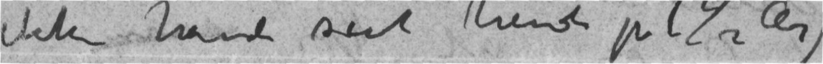ikke havde seet hende på 1 ½ År)
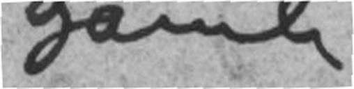gamle
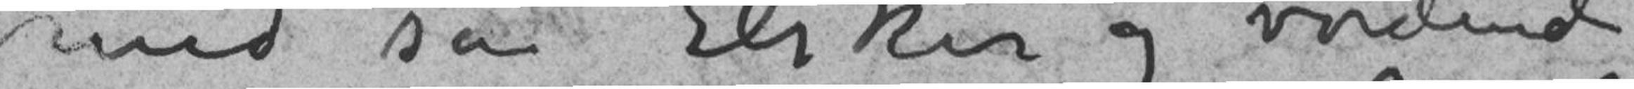med sin Elsker og vordende
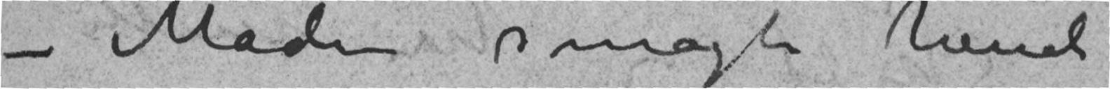– Maden smagte hende
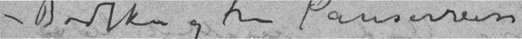– Bødtker og Frue Pariserreise
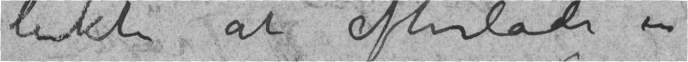likte at efterlade en
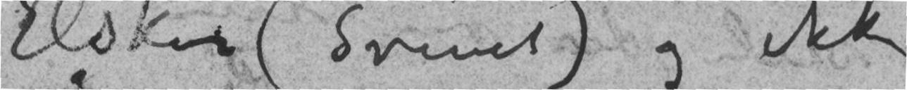Elsker (Svinet) og ikke
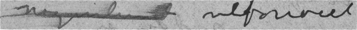nogenlunde velfornøiet
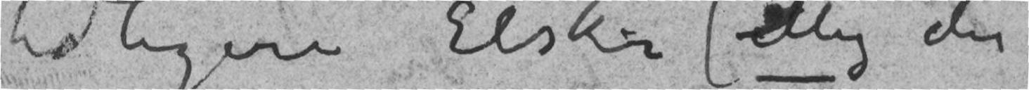tidligere Elsker (Mig der
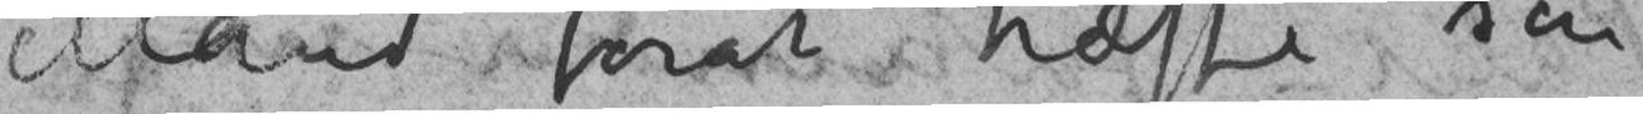Mand forat træffe sin
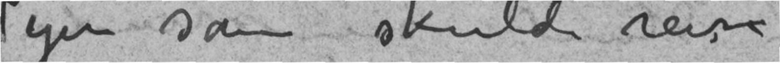Pigen som skulde reise
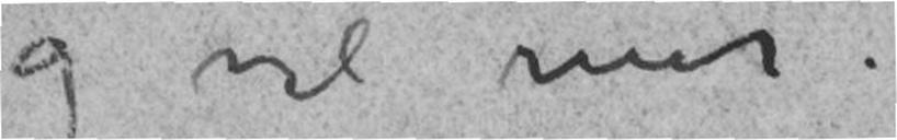og vil mer.
